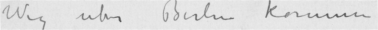Weg uber Berlin kommen
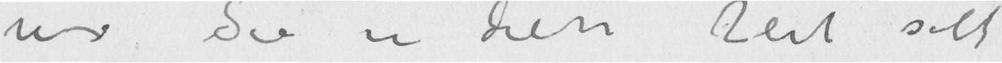wo Sie in den Zeit sich
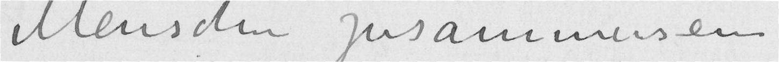Menschen zusammensein
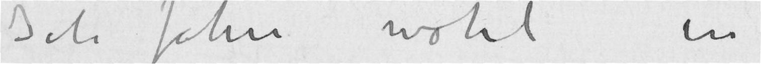Ich fahre wohl in
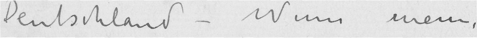Deutschland – Wenn meine
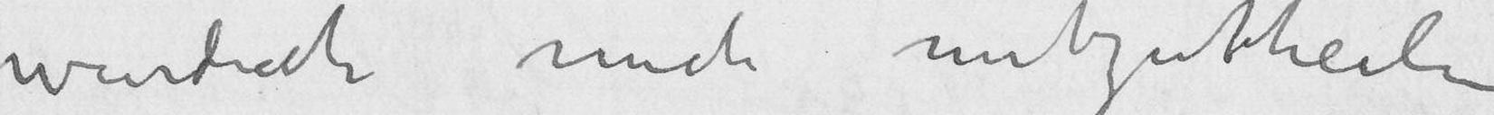wurdich mich mitzutheilen
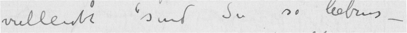vielleicht sind Sie so liebens-
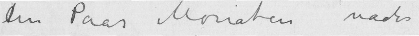ein Paar Monaten nach
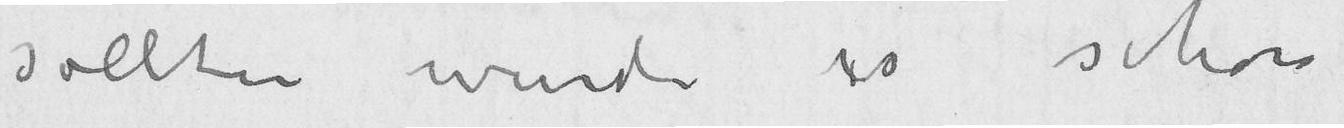sollten wurde so schon
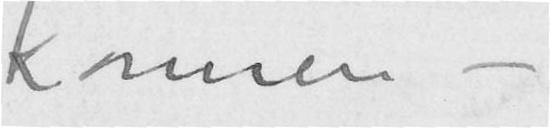konnen –
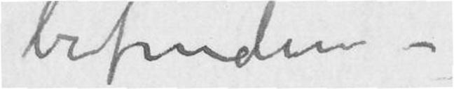befinden –
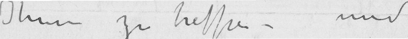Ihnen zu treffen – und
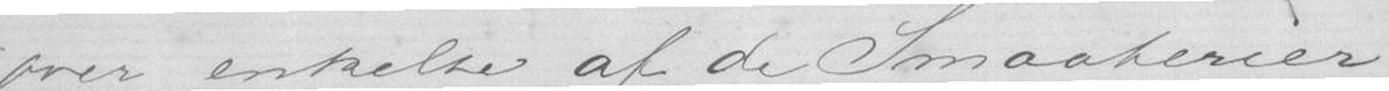over enkelte af de Smaaterier
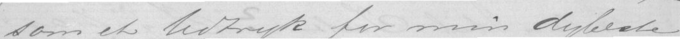som et Udtryk for min dybeste
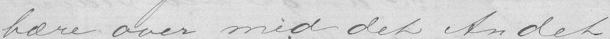bære over med det Andet,
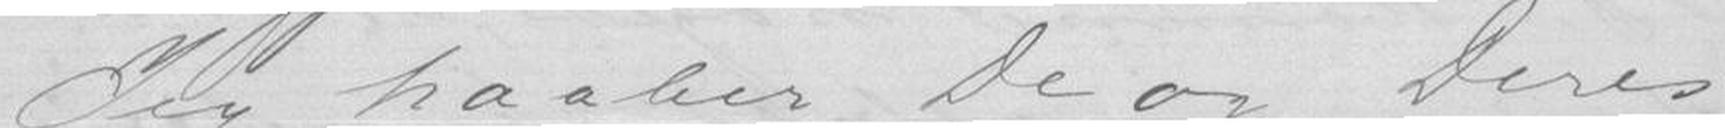Jeg haaber De og Deres
